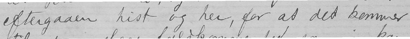eftergaaen hist og her, for at det kommer
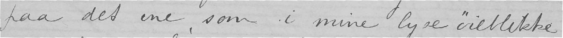paa det ene, som i mine lyse øieblikke
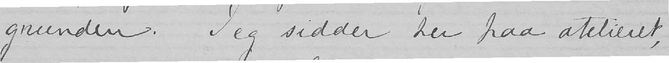grunden. Jeg sidder her paa atelieret,
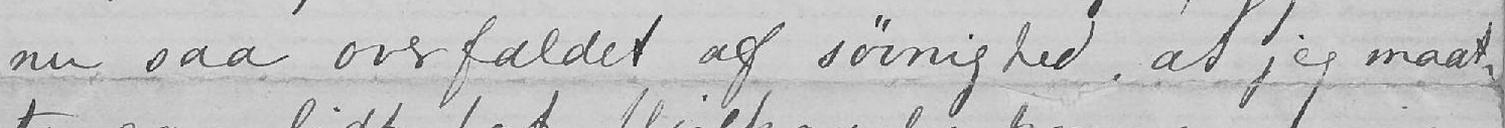nu saa overfaldet af søvnighed, at jeg maat-
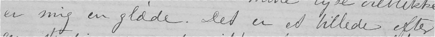er mig en glæde. Det er et billede efter
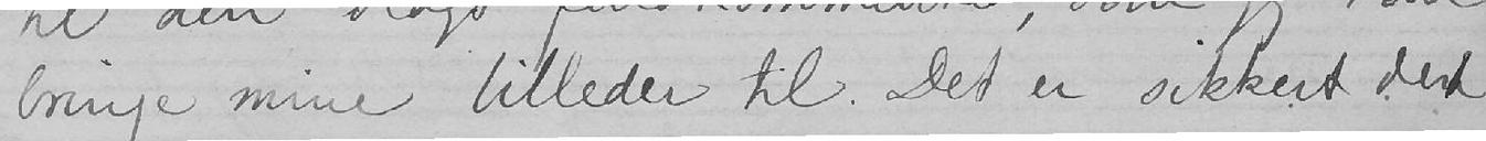bringe mine billeder til. Det er sikkert det
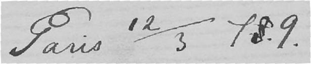Paris 12/3 79
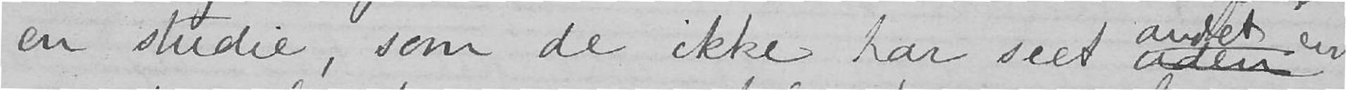en studie, som de ikke har seet anden
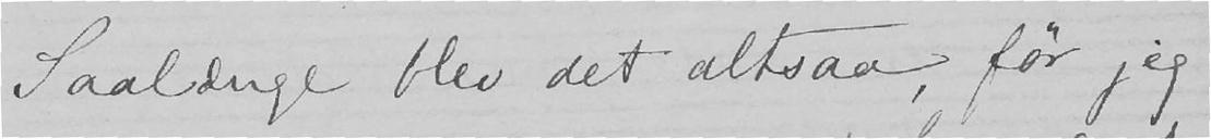Saalænge blev det altsaa, før jeg
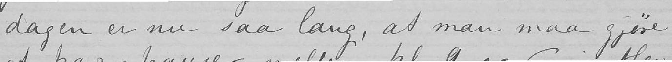dagen er nu saa lang, at man maa gjøre
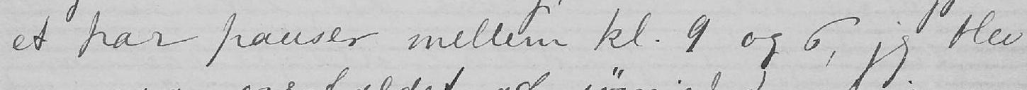et par pauser mellem kl. 9 og 6, jeg blev
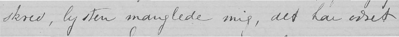skrev, lysten manglede mig, det har været
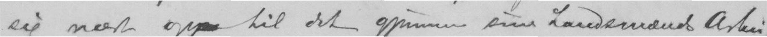sig nært opp til det gjennem sine Landsmænds Arbei-
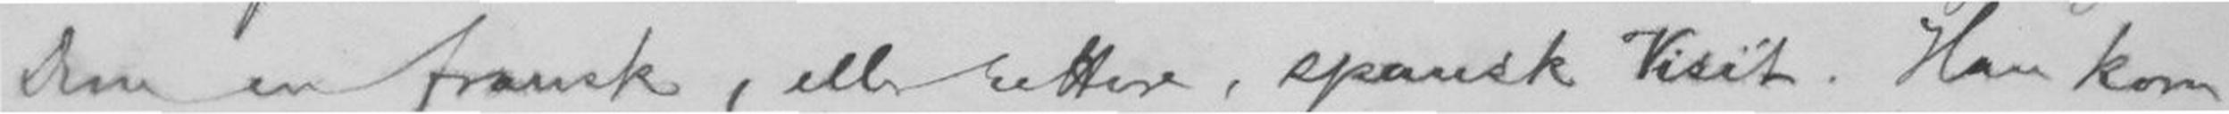Dem en fransk, eller rettere, spansk Visit. Han kom-
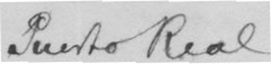Puerto Real
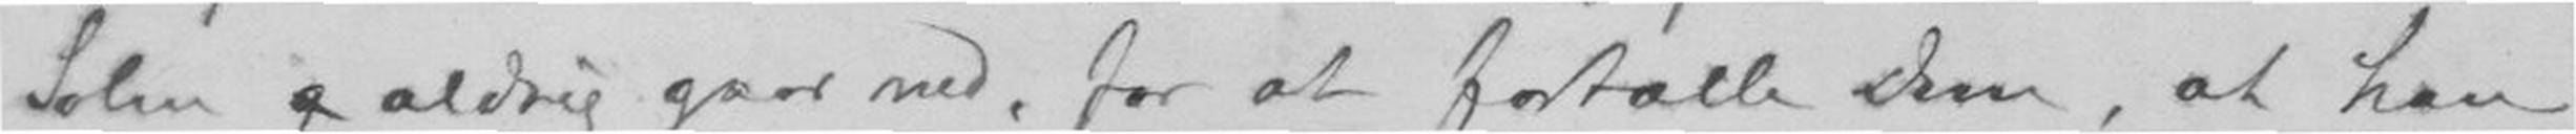Solen g aldrig gaar ned, for at fortælle Dem, at han
